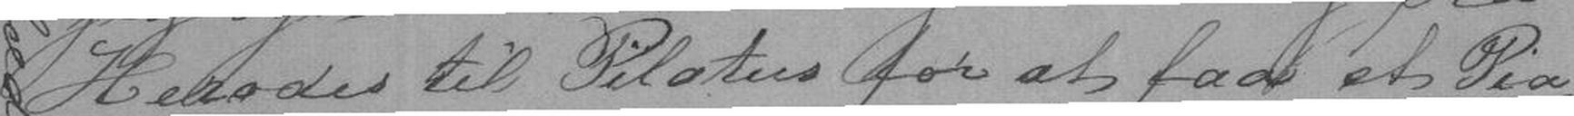Herodes til Pilatus for at faa et Pia-
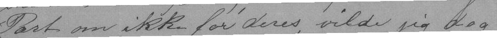Part om ikke for deres vilde jeg dog
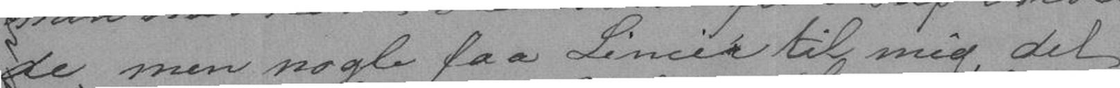de; men nogle faa Linier til mig, det
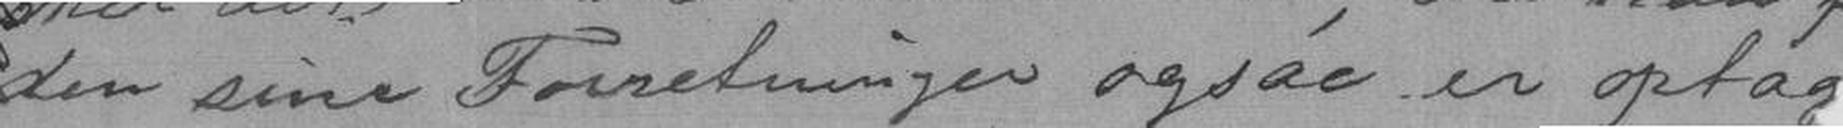den sine Forretninger ogsaa er optag
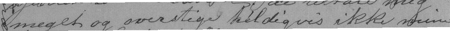meget og overstege heldigvis ikke mine
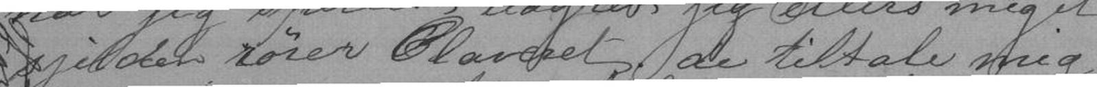sjelden rører Claveret; de tiltale mig
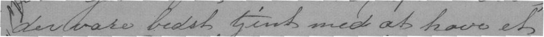der vare bedst tjent med at have et
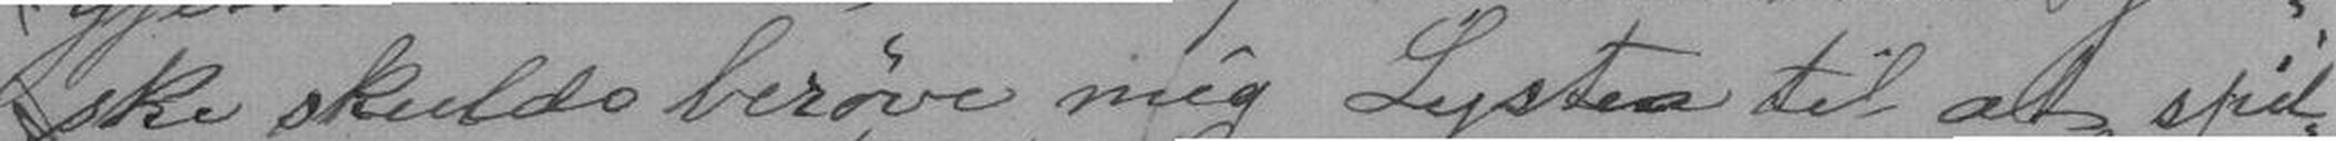ske skulde berøve mig Lysten til at spil-
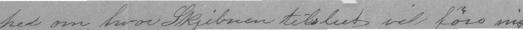hed om hvor Skjæbnen tilslut vil føre mig
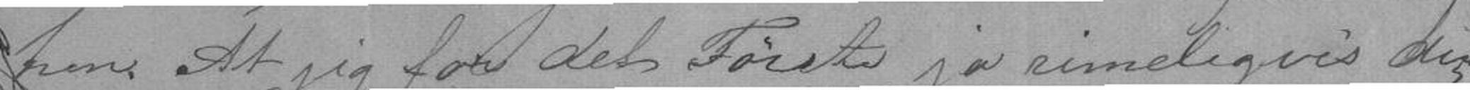hen. At jeg for det Første, ja rimeligvis den
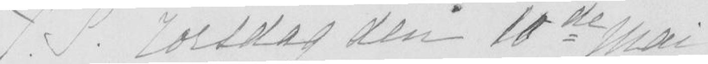P.S. Torsdag den 18de Mai
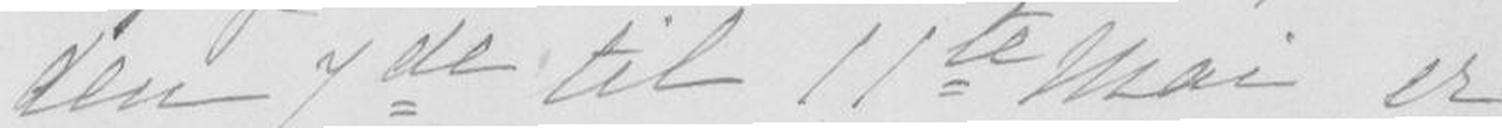den 7de til 11te Mai er
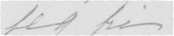jeg fri -.
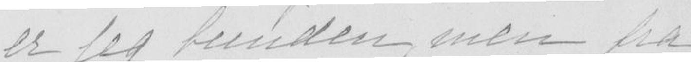er jeg bunden, men fra
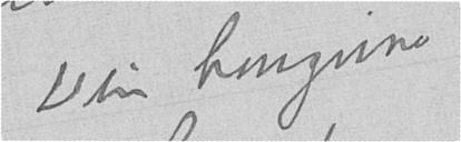Din hengivne
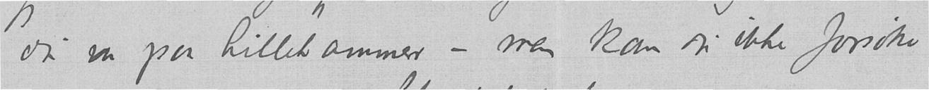du var paa Lillehammer - men kan du ikke forsøke
